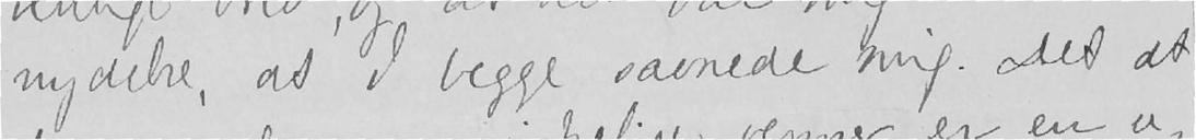nydelse, at I begge savnede mig. Det at
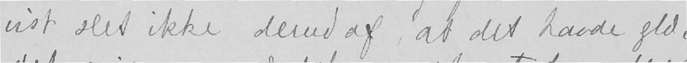vist slet ikke derudaf, at det havde glæ-
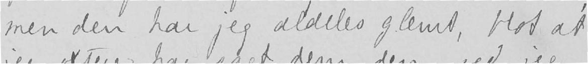men den har jeg aldeles glemt, blot at
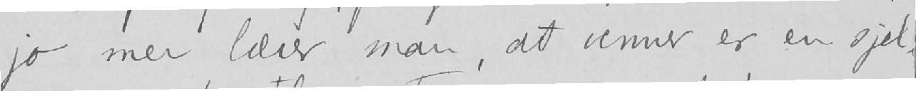jo mer lærer man, at venner er en sjel-
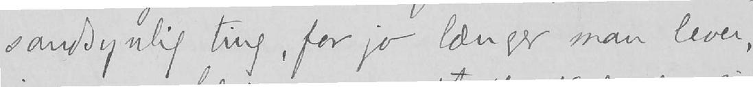sandsynlig ting, for jo længer man lever,
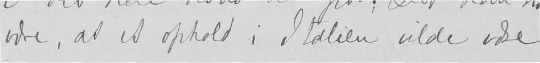være, at et ophold i Italien vilde være
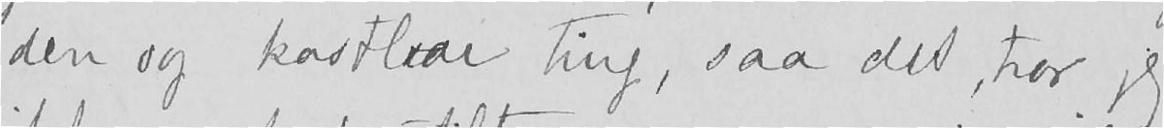den og kostbar ting, saa det, tror jeg
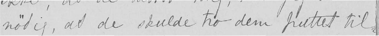nødig, at de skulde tro dem puttet til-
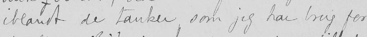iblandt de tanker, som jeg har brug for
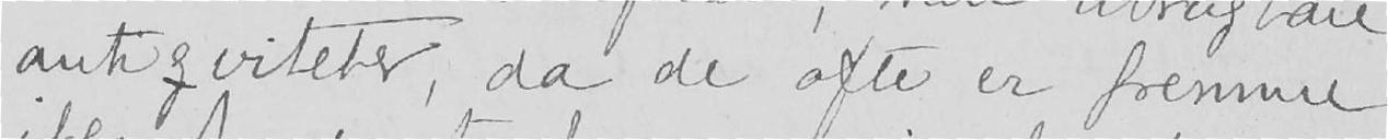antiqviteter, da de ofte er fremme
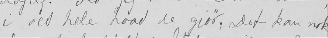i det hele hvad de gjør; Det kan nok
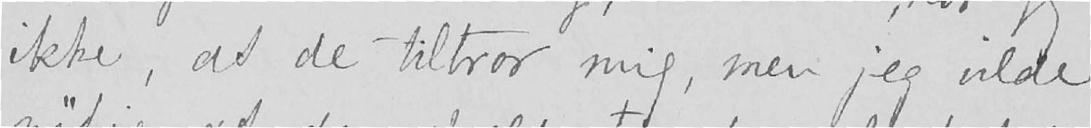ikke, at de tiltror mig, men jeg vilde
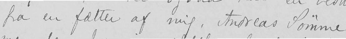fra en fætter af mig, Andreas Sømme,
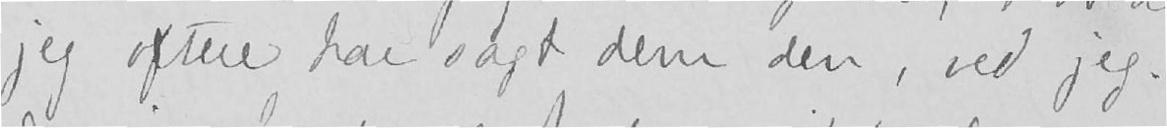jeg oftere har sagt dem den, ved jeg.
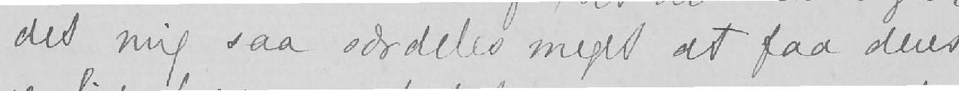det mig saa særdeles meget at faa deres
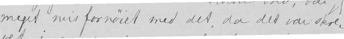meget misfornøiet med det, da det var skre-
